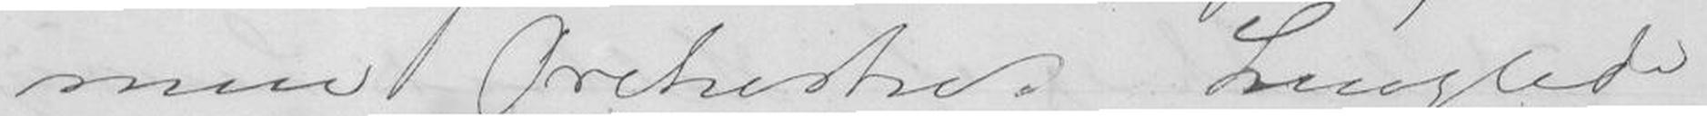men Orchestret henglede
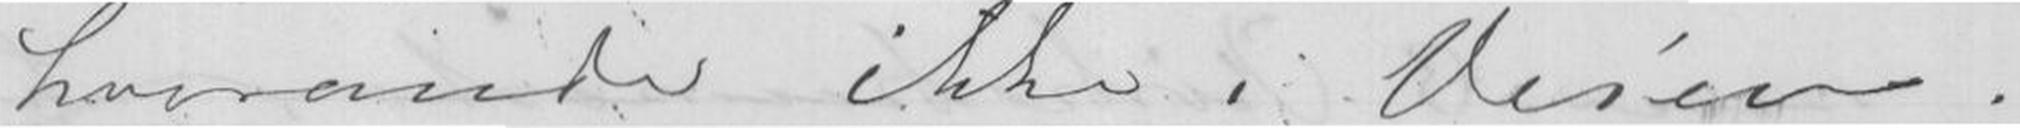hverandre ikke i Veien
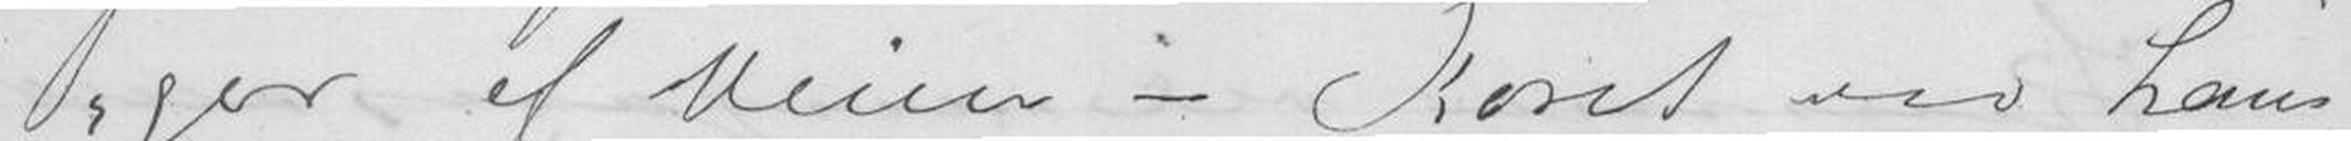ger af Veien. Koret var hans
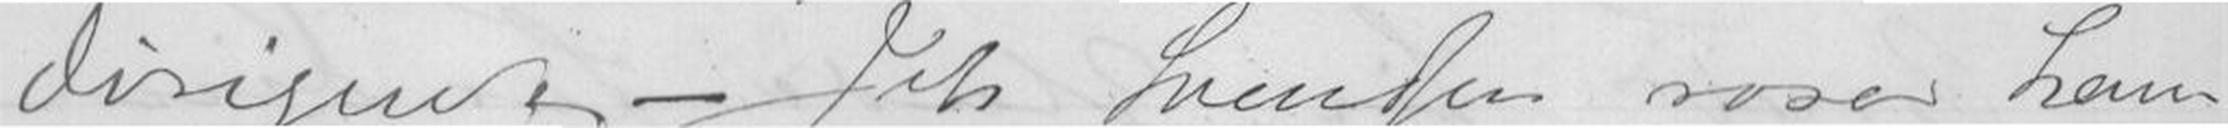dirigent- Joh Svendsen roser ham
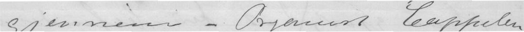gjennem - Organist Cappelen
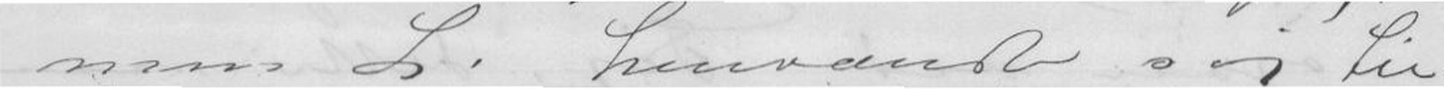men L. henvænde sig til
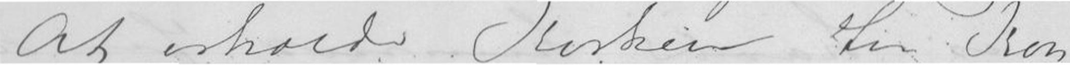At erholde Kirken til Kon
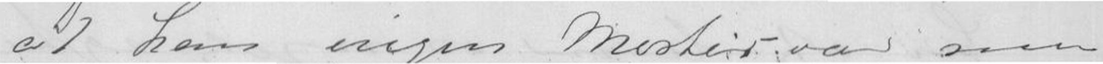at han ingen Mester var som
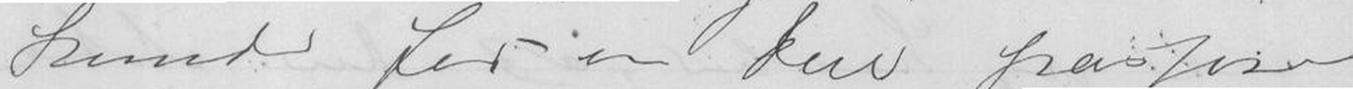kunde for en Deel passere
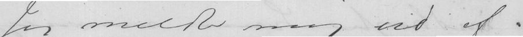Jeg meldte mig ud af.
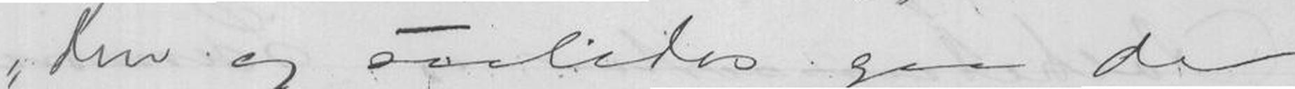den og saaledes gaa de
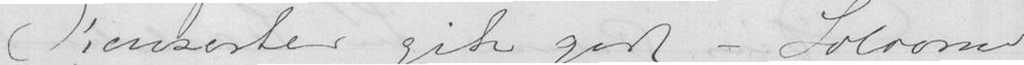Konserter gik godt - Soloerne
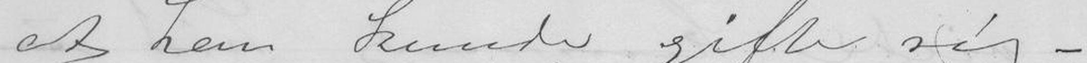at han kunde gifte sig -
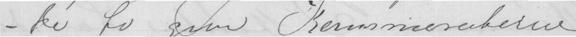- De to give Kammeraterne
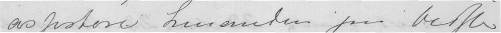assistere hinanden paa bedste
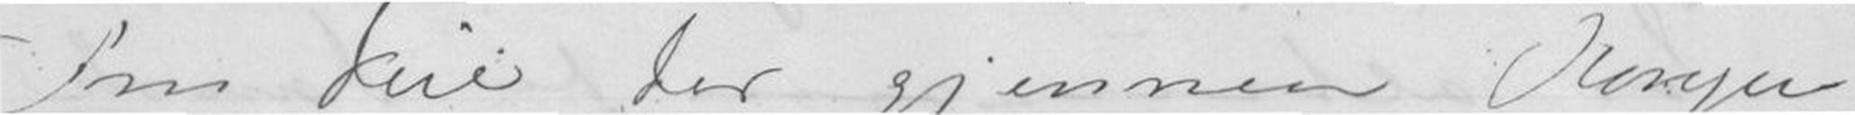Fru Due der gjennem Kongen
